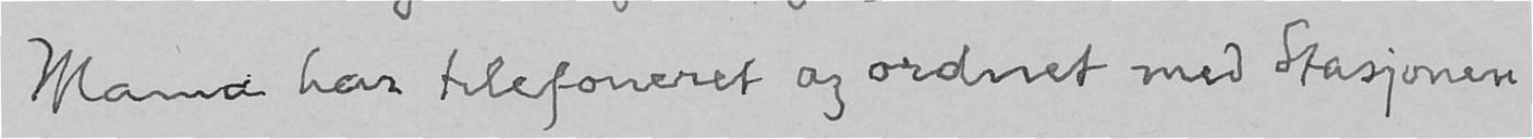Mama har telefoneret og ordnet med Stasjonen
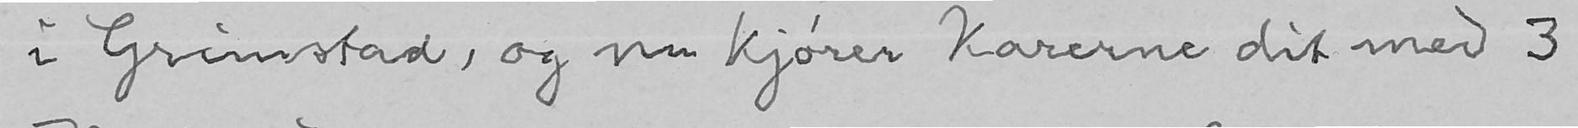i Grimstad, og nu kjører Karerne dit med 3
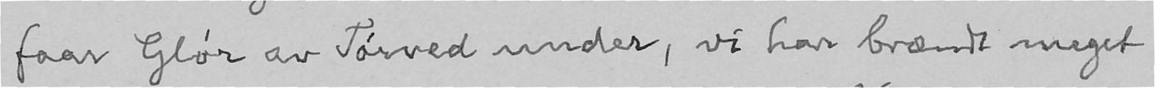faar Glør av Tørved under, vi har brændt meget
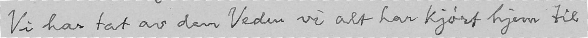Vi har tat av den Veden vi alt har kjørt hjem til
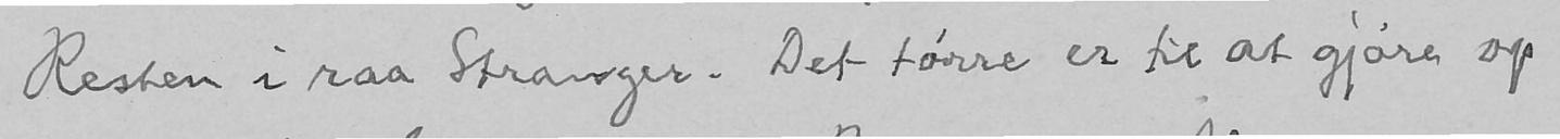Resten i raa Stranger. Det tørre er til at gjøre op
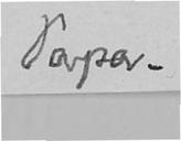Papa.
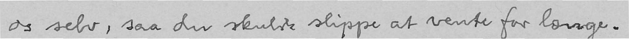os selv, saa du skulde slippe at vente for længe.
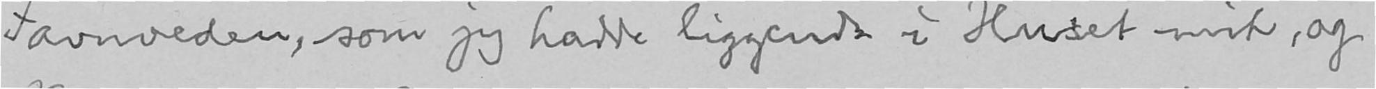Favnveden, som jeg hadde liggende i Huset mit, og
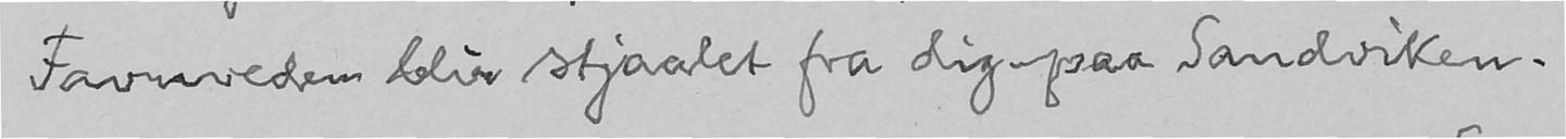Favnveden blir stjaalet fra dig paa Sandviken.
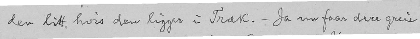den litt hvis den ligger i Træk. - Ja nu faar dere greie
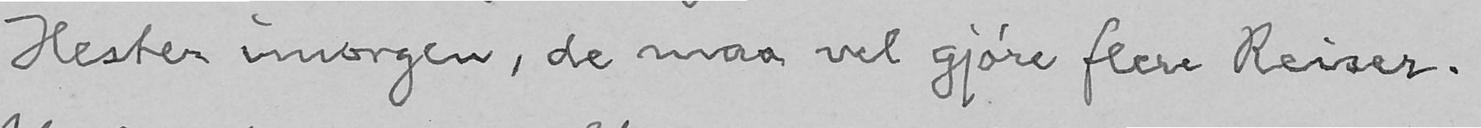Hester imorgen, de maa vel gjøre flere Reiser.
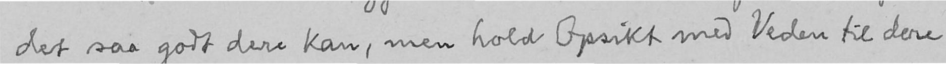det saa godt dere kan, men hold Opsikt med Veden til dere
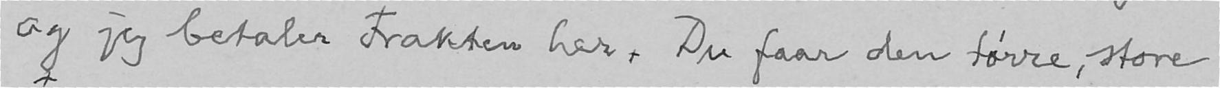og jeg betaler Frakten her. Du faar den tørre, store
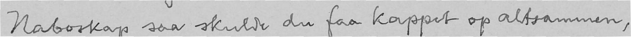Naboskap saa skulde du faa kappet op altsammen,
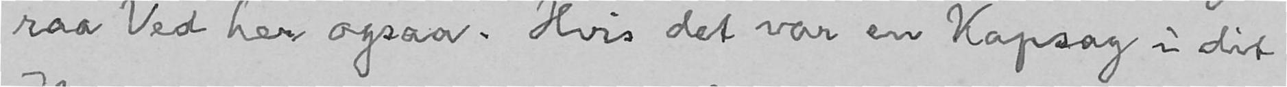raa Ved her ogsaa. Hvis det var en Kapsag i dit
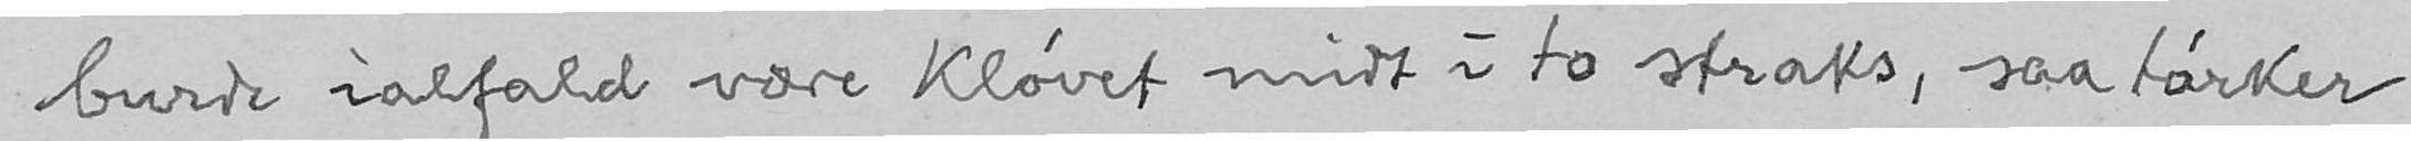burde ialfald være kløvet midt i to straks, saa tørker
